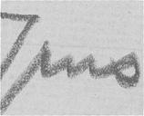Jens
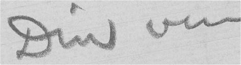Din ven
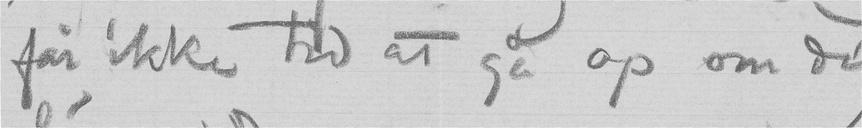får ikke tid at gå op om dig
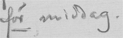før middag.
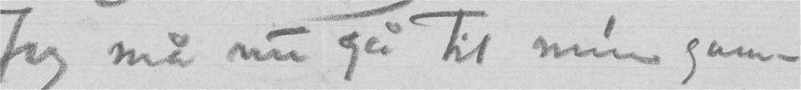Jeg må nu gå til min gam-
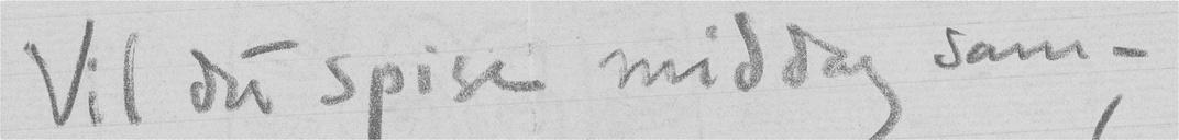Vil du spise middag sam-
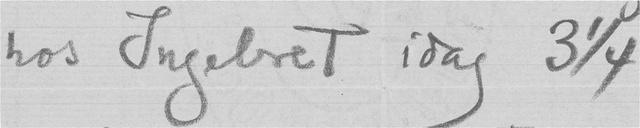hos Ingebret idag 3 ¼
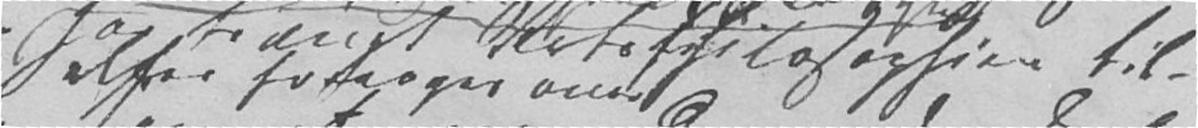har trængt Retsphilosophien til-
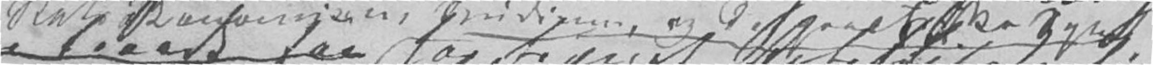Rat konstringen, Sindige og spanske synt
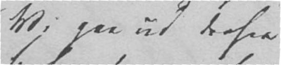Vi gaa ud derfra
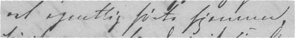ret egentlig hørte hjemme,
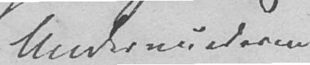Undervurderen
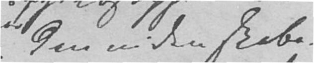den videnskabe-
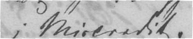i Miscredit.
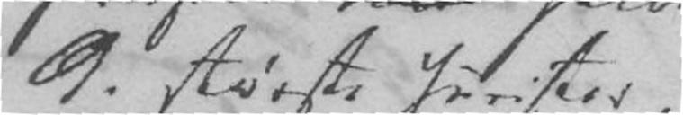de største Jurister,
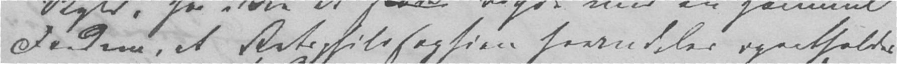Fordom, et Retsphilosophien fremdeles opretholdes
